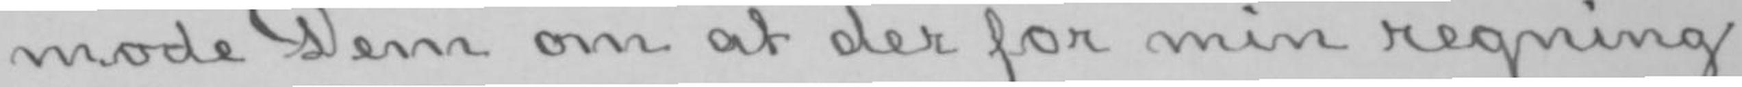mode Dem om at der for min regning
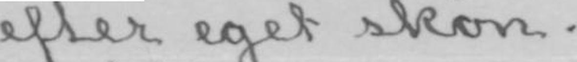efter eget skøn.
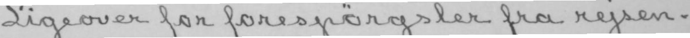Ligeover for forespørgsler fra rejsen-
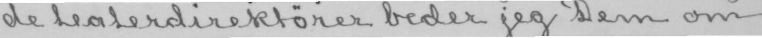de teaterdirektører beder jeg Dem om
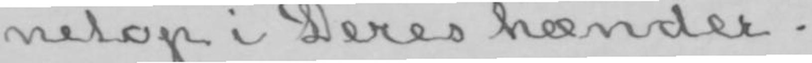netop i Deres hænder.
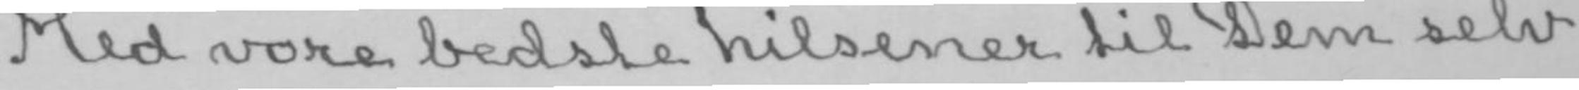Med vore bedste hilsener til Dem selv
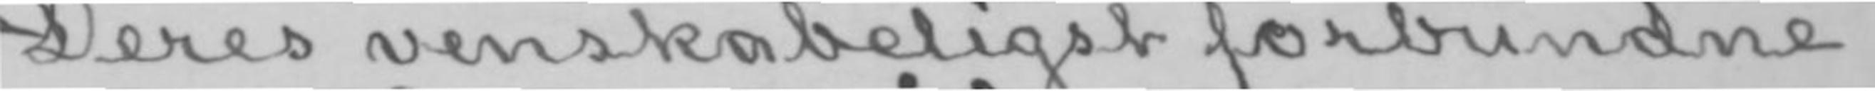Deres venskabeligst forbundne
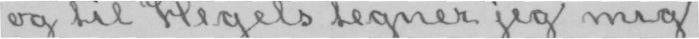og til Hegels tegner jeg mig
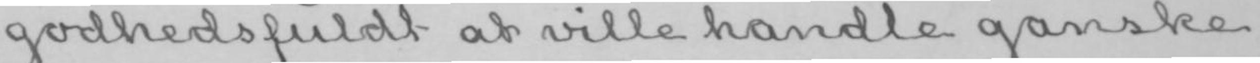godhedsfuldt at ville handle ganske
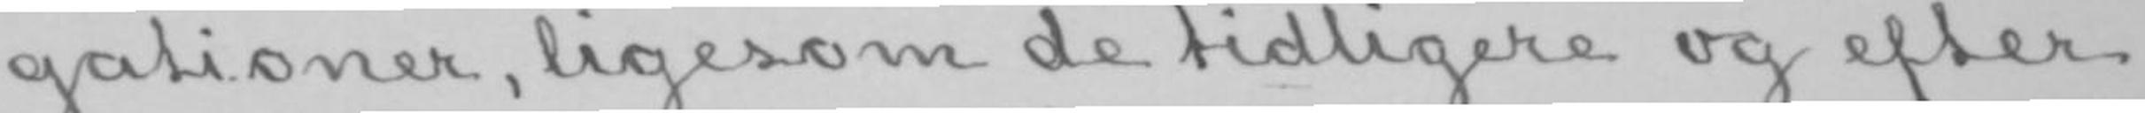gationer, ligesom de tidligere og efter
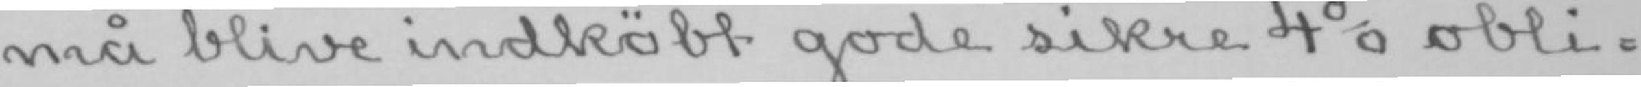må blive indkøbt gode sikre 4% obli-
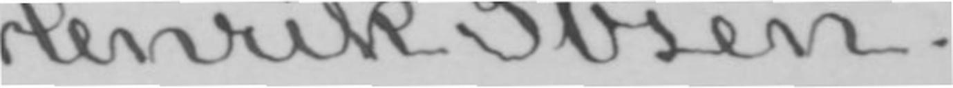Henrik Ibsen.
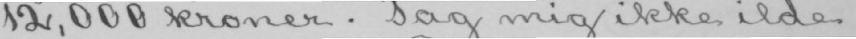12,000 kroner. Tag mig ikke ilde
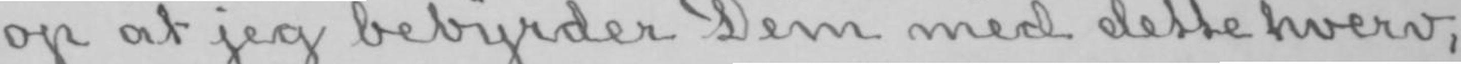op at jeg bebyrder Dem med dette hverv,
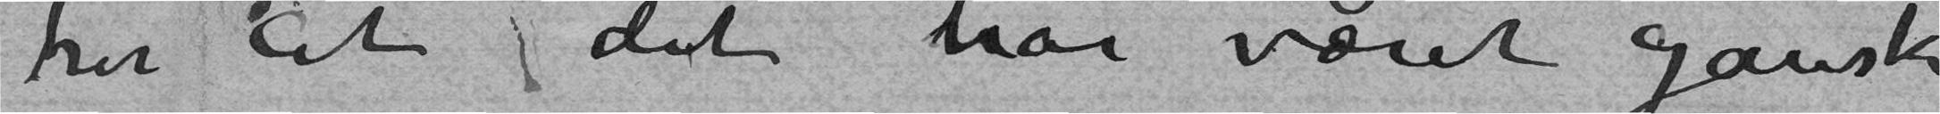tror at det har været ganske
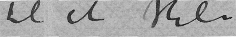til et Hele
